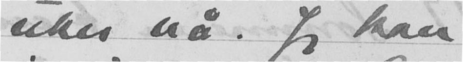uker nå. Jeg kan
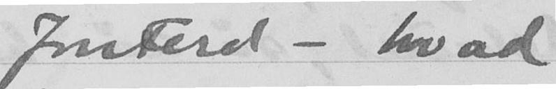Jonferd - hvad
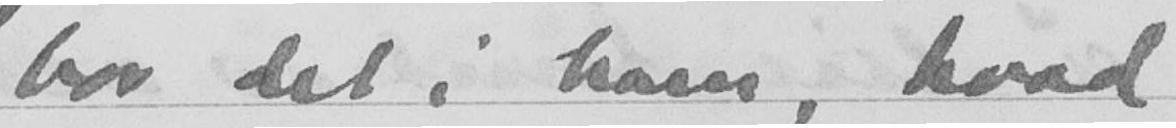bor det i ham, hvad
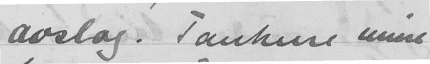avslag. Tankene mine
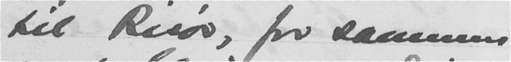til Risør, for sammen
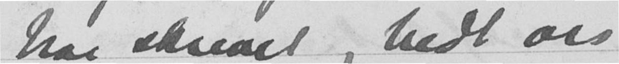har skrevet og bedt oss
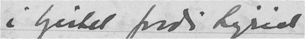i hjertet fordi Sigrid
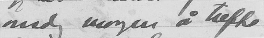onsdag morgen å treffe
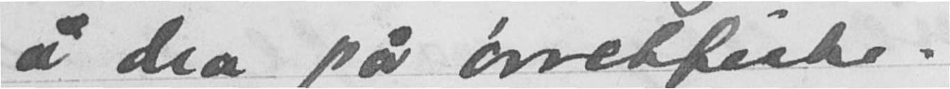å dra på ørretfiske.
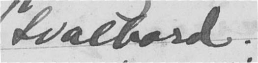Svalbard. -
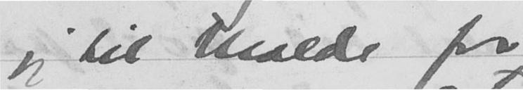jeg til Molde for
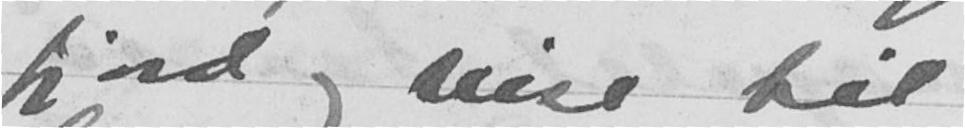fjord og reise til
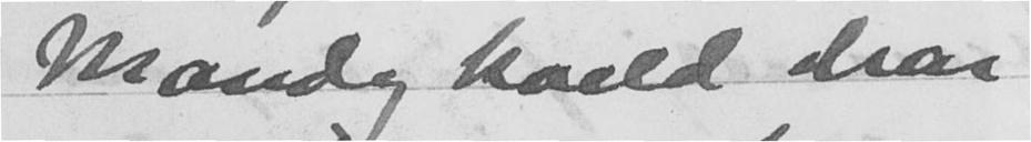Mandag kveld drar
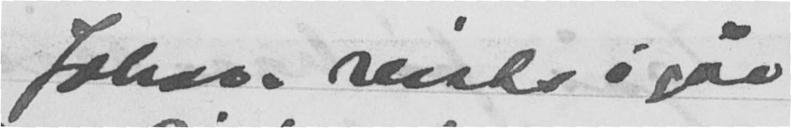Johan reiste igår
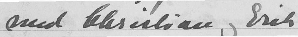med Christian og Erik
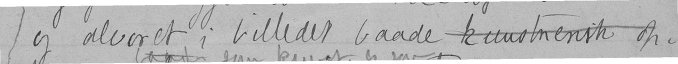og alvoret i billedet baade kunstnerisk op-
